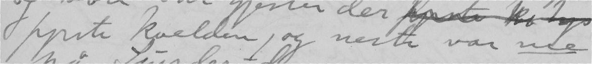Fyrste kvelden, og neste var me
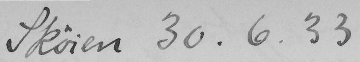Sköien 30.6.33
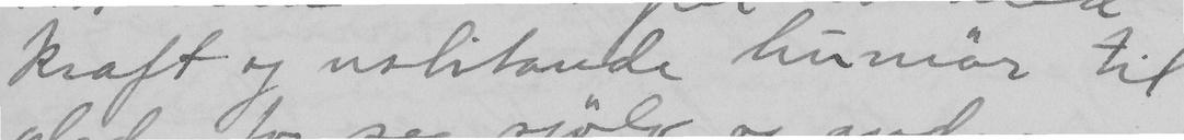kraft og uslitande humör til
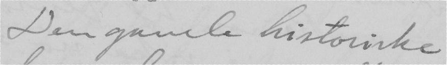Den gamle historiske
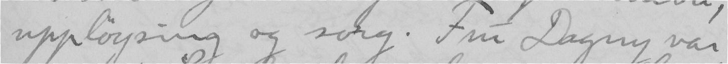upplöysing og sorg. Fru Dagny var
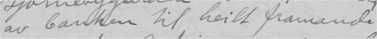av banken til heilt framande
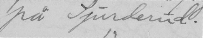på Sjurderud.
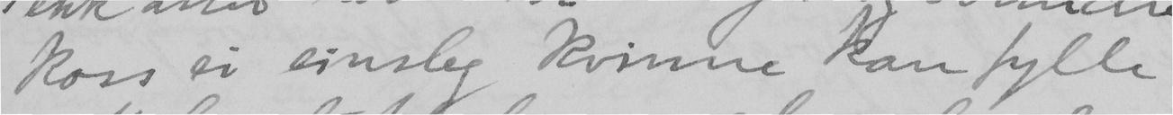koss ei einsleg kvinne kan fylle
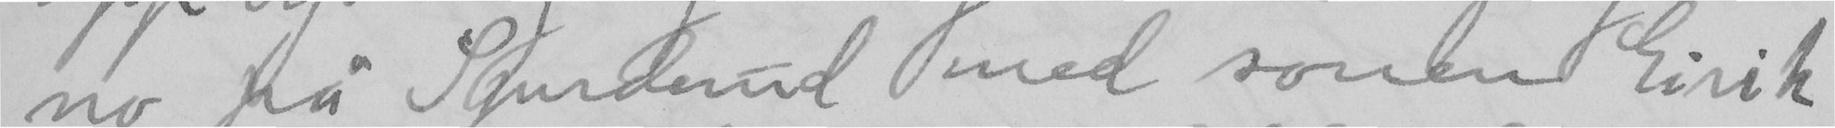no på Sjurderud med sonen Eirik
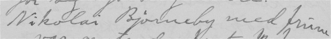Nikolai Björneby med frue
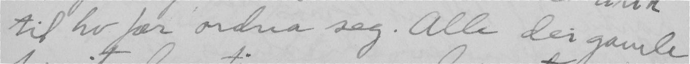til ho fær ordna seg. Alle dei gamle
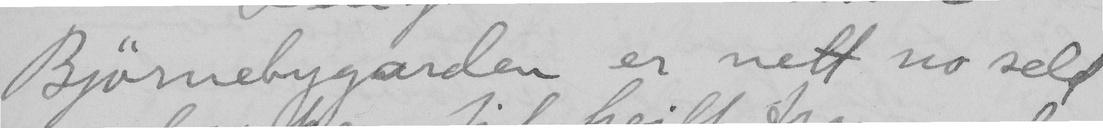Björnebygarden er nett no seld
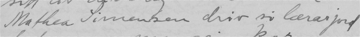Mathea Simensen driv si lærarjord
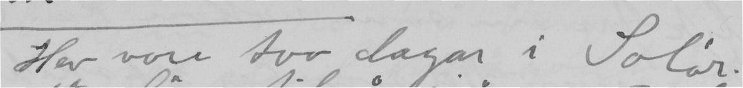Hev vore tvo dagar i Solöør.
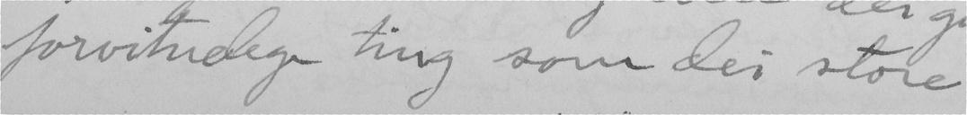forvitnelege ting som dei store
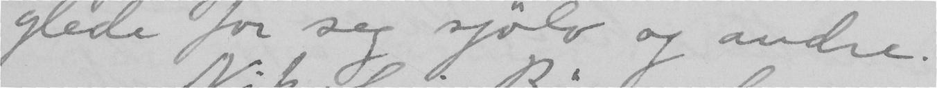glede for seg sjölv og andre.
